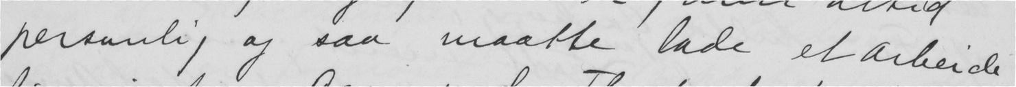personlig og saa maatte lade et arbeide
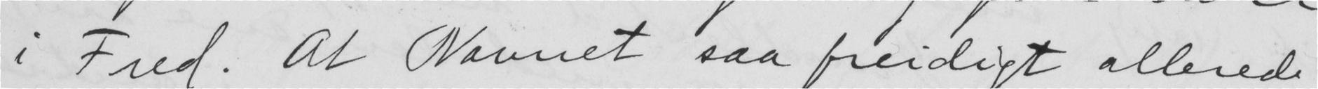i Fred. At Navnet saa freidigt allerede
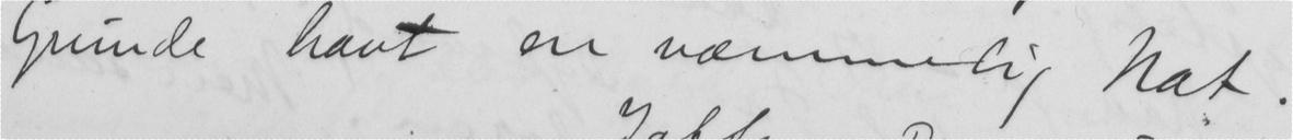Grunde havt en væmmelig Nat.
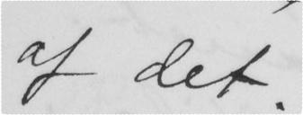af det
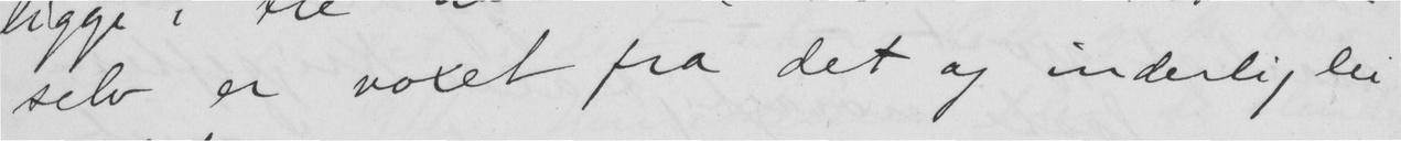selv er voxet fra det og inderlig lei
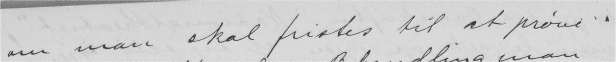om man skal fristes til at prøve
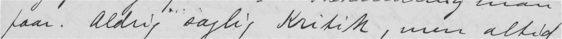faar. Aldrig saglig Kritik, men altid
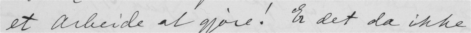et Arbeide at gjøre! Er det da ikke
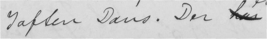Iaften Dans. Der
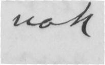nok
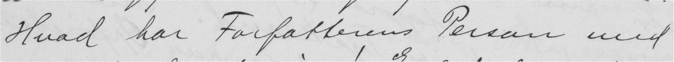Hvad har Forfatterens Person med
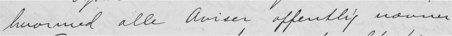hvormed alle aviser offentlig nævner
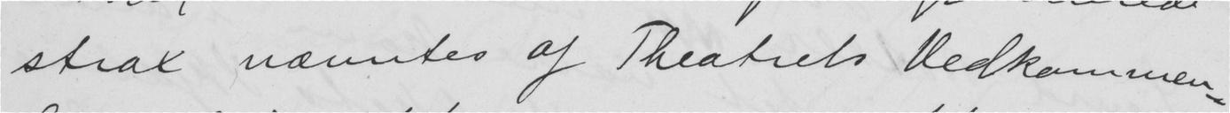strax nævntes af Theatrets Vedkommen-
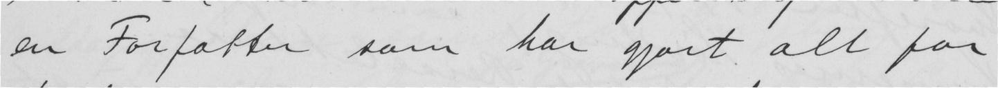en Forfatter som har gjort alt for
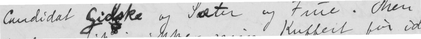Candidat Gidske og Sæter og Frue. Men
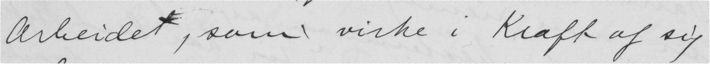Arbeidet, som virke i Kraft af sig
